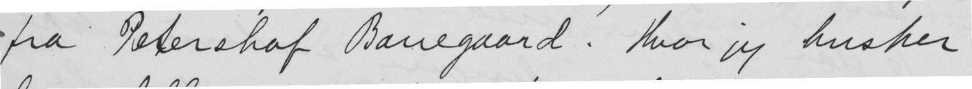fra Petershof Banegaard. Hvor jeg husker
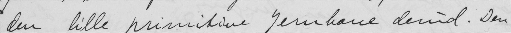den lille primitive Jernbane derud. Den
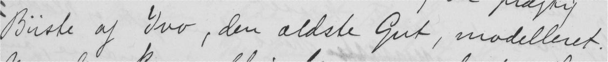Buste af Ivo, den ældste Gut, modelleret.
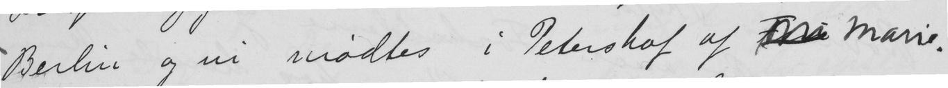Berlin og vi mødtes i Petershof af  Marie.
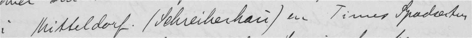i Mitteldorf. (Schreiberhau) en Times Spadsertur
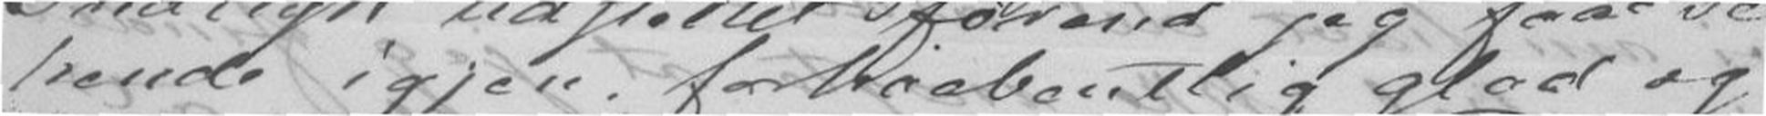hende igjen forhaabentlig glad og
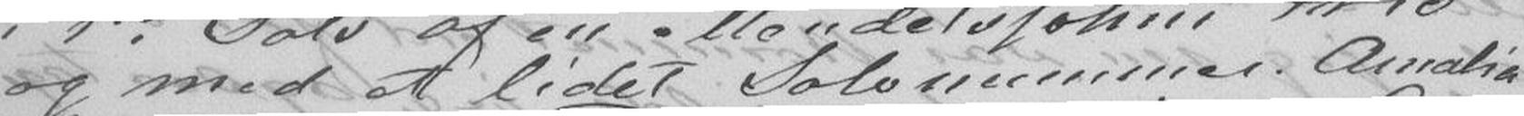og med et lidet Solonummer. Amalia
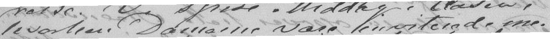hvorhen Damerne være inviterede me-
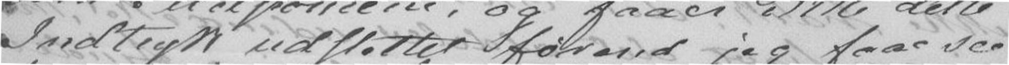Indtryk udslettet førend jeg faae see
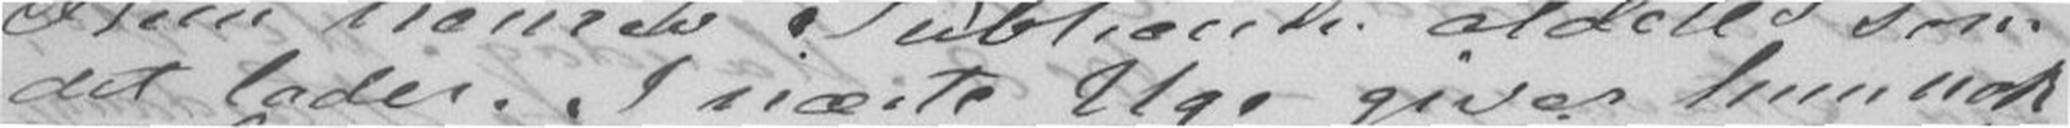det lader. J næste Uge giver hun nok
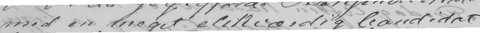med en meget elskværdig Candidat
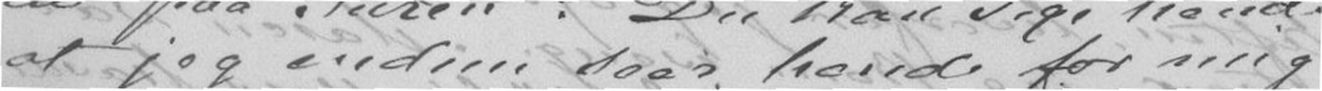at jeg endnu seer hende for mig
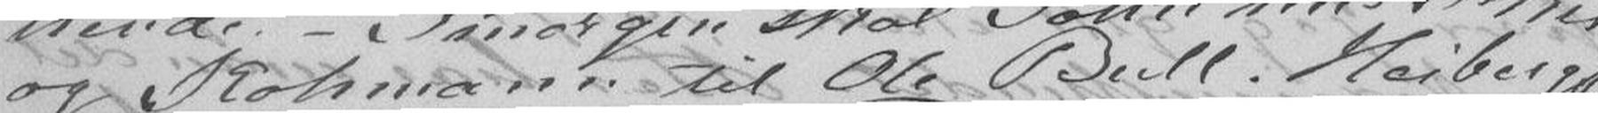og Kohmann til Ole Bull. Heibergs
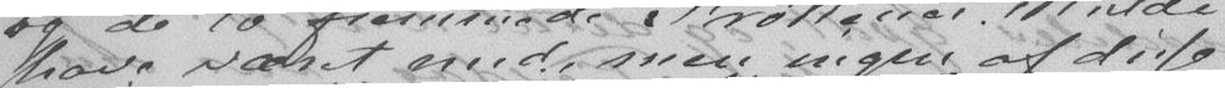have været med, men ingen af disse
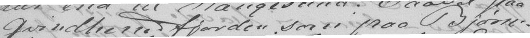Qvindherredfjorden som paa Bjørne-
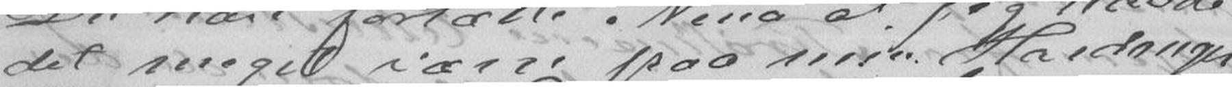det meget værre paa min Hardanger
